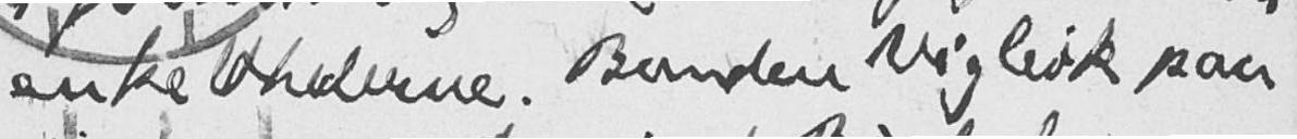enkelthederne. Bonden Vigleik paa
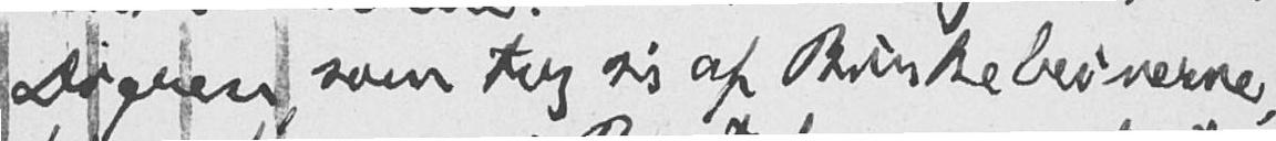Digren, som tog sig af Birkebeinerne,
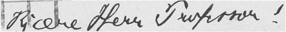Kjære Herr Professor!
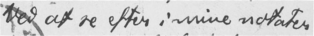Ved at se efter i mine notater
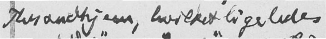Throndhjem, hvilket ligeledes
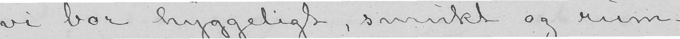vi bor hyggeligt, smukt og rum-
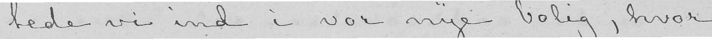tede vi ind i vor nye bolig, hvor
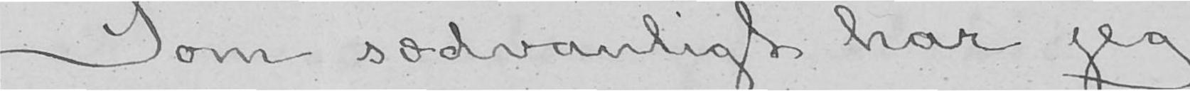Som sædvanligt har jeg
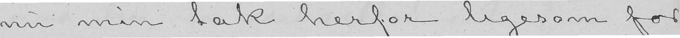nu min tak herfor ligesom for
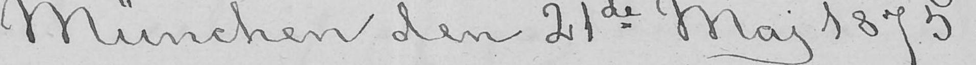München den 21de Maj 1875.
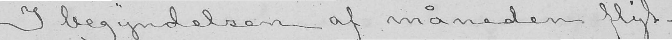I begyndelsen af måneden flyt-
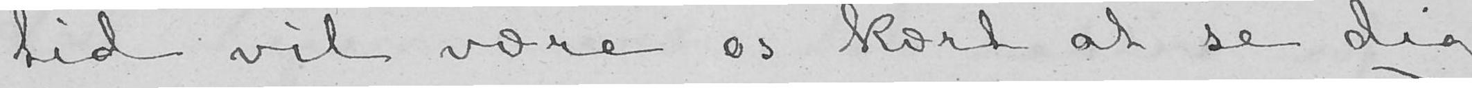tid vil være os kært at se dig
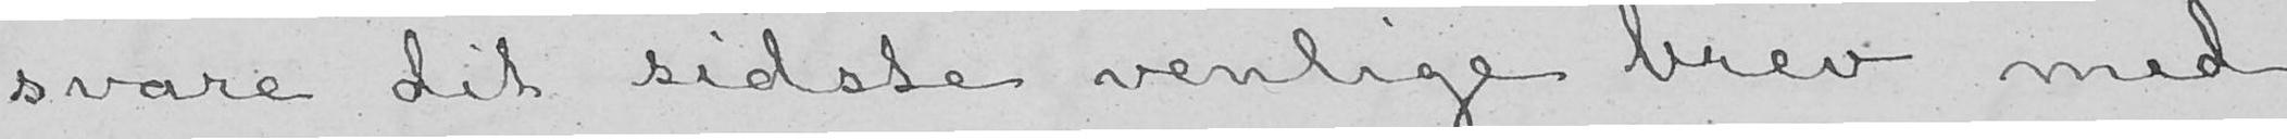svare dit sidste venlige brev med
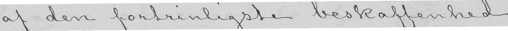af den fortrinligste beskaffenhed
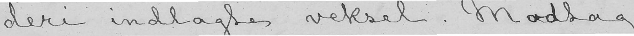deri indlagte veksel. Modtag
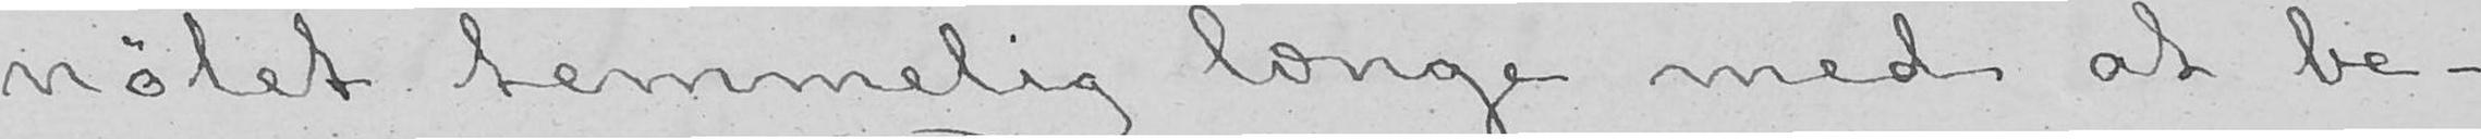nølet temmelig længe med at be-
